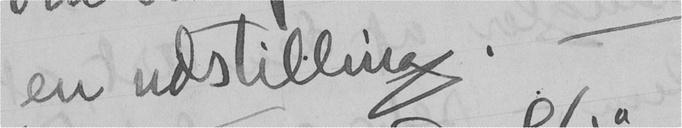en udstilling. -
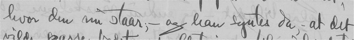hvor den nu staar; - og han syntes da, - at det
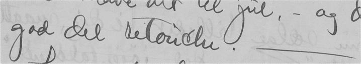god del retouche. -
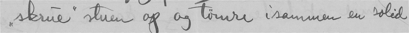"skrue" stuen op og tømre isammen en solid
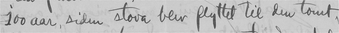100 aar, siden stova blev flyttet til den tomt,
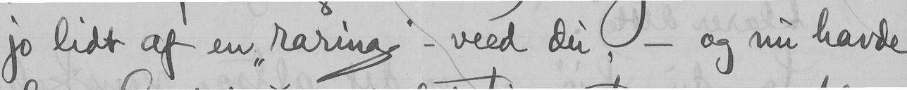jo lidt af en "raring" - veed du, - og nu havde
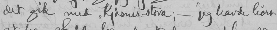det gik med "Kjøsnes-stova; - jeg havde hørt
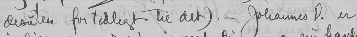desuten for tidligt til det). - Johannes D. er
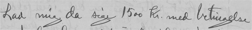Lad mig da sige 1500 Kr. med betingelse
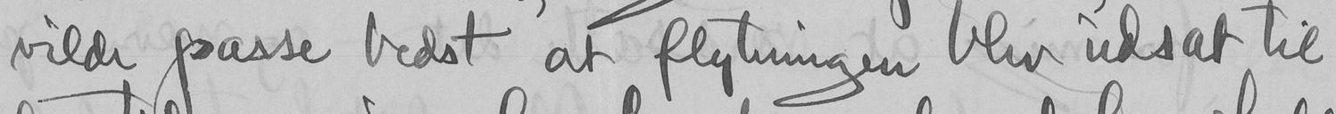vilde passe bedst at flytningen blev udsat til
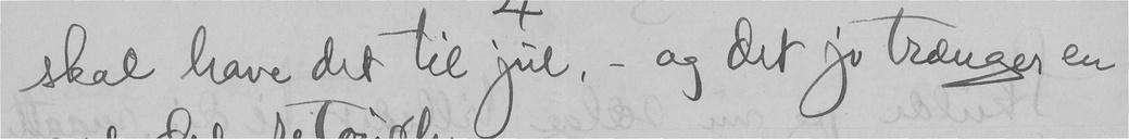skal have det til jul, - og det jo trænger en
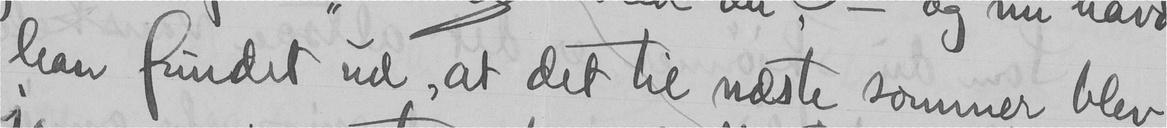han fundet ud, at det til næste sommer blev
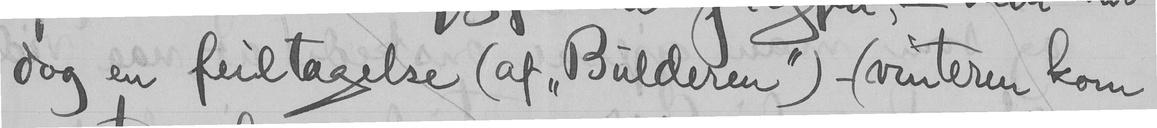dog en feiltagelse (af "Bulderen") - (vinteren kom
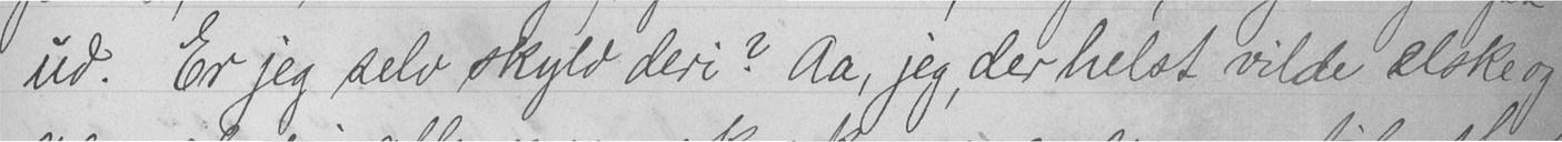ud. Er jeg selv skyld deri? Aa, jeg, der helst vilde elske og
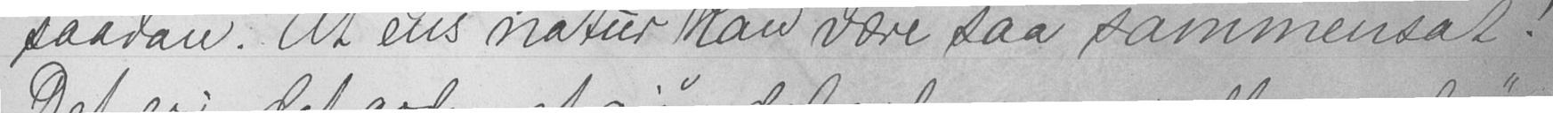saadan. At ens natur kan være saa sammensat!
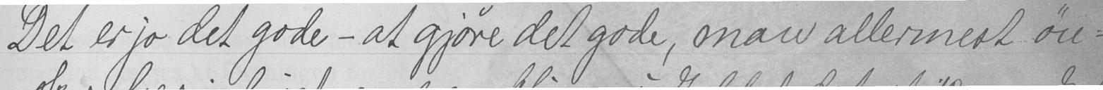Det er jo det gode - at gjøre det gode, man allermest øn-
